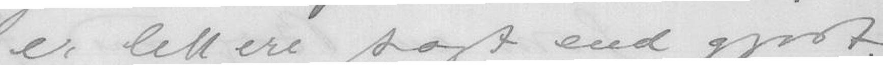er lettere sagt end gjort.
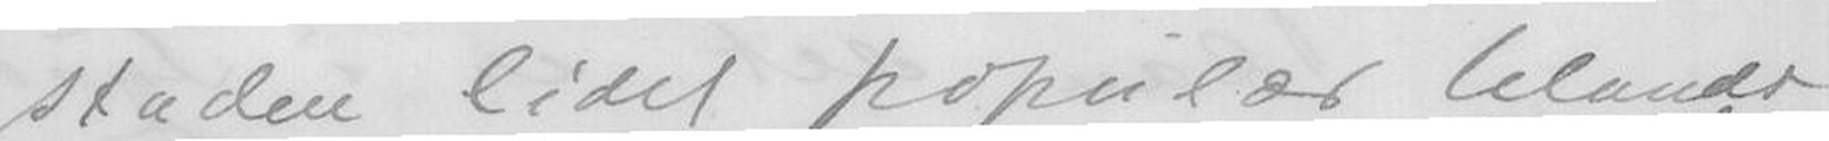staden lidet populær blandt
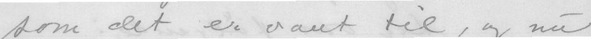som det er vant til, og nu
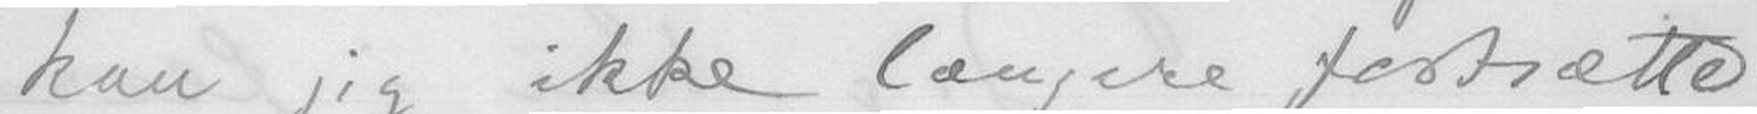kan jeg ikke længere fortsætte
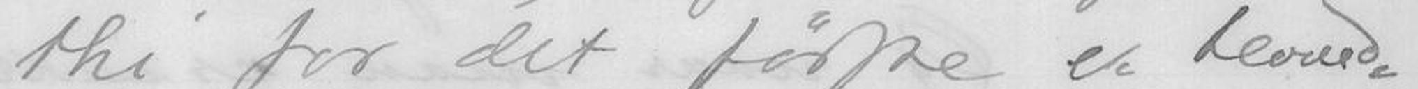thi for det første er Hoved-
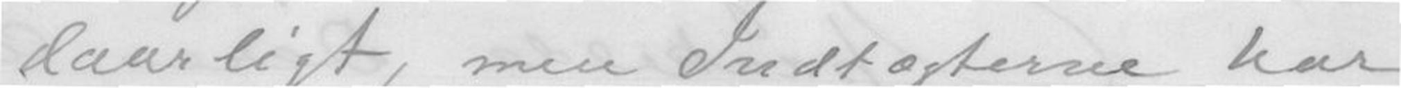daarligt, men Indtægterne har
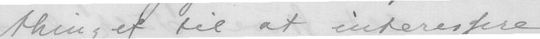thinget til at interessere
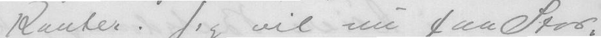Kanter. Jig vil nu faa Stor-
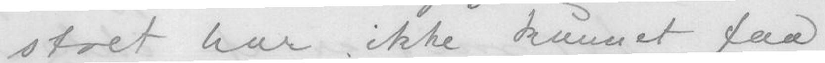stret har ikke kunnet faa
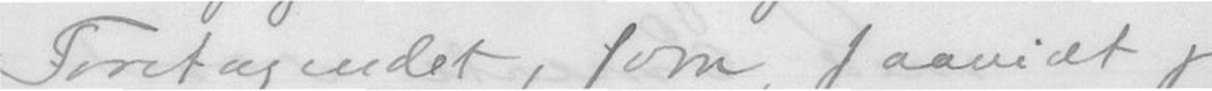Foretagendet, som, saavidt jeg
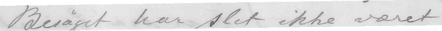Besøget har slet ikke været
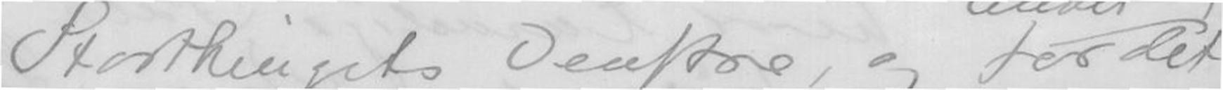Storthingets Venstre, og for det
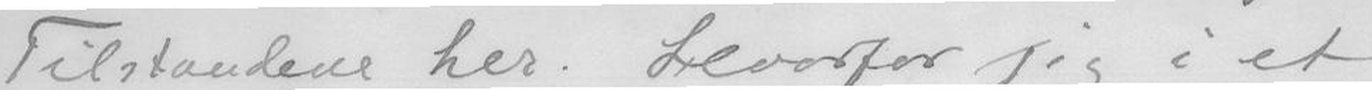Tilstandene her. Hvorfor jig i et
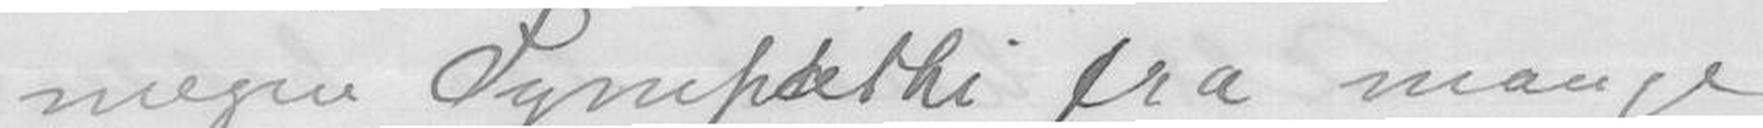megen Sympathi fra mange
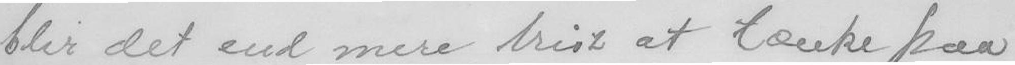Blir det end mere trist at tænke paa
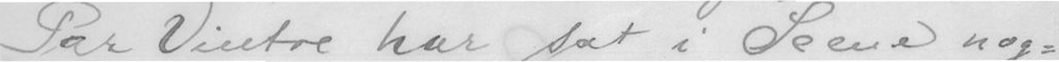Par Vintre har sat i Scene nog-
